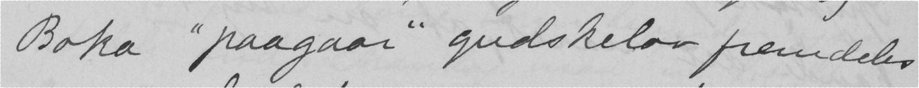Boka "paagaar" gudskelov fremdeles
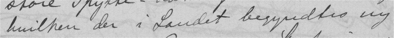hvilken der i Landet begyndtes ny
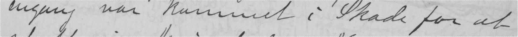engang var kommet i Skade for at
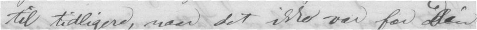til tidligere, naar det ikke var for Din
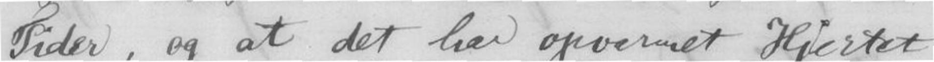Tider, og at det har opvarmet Hjertet
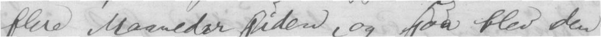flere Maaneder siden, og saa blev den
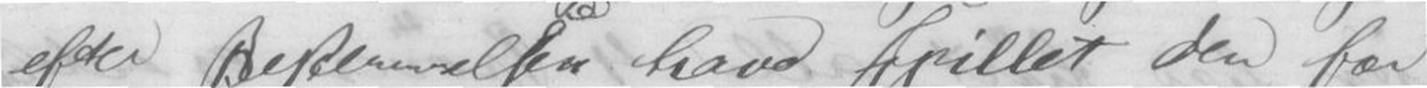efter Bestemmelsen have spillet den for
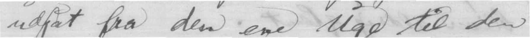udsat fra den ene Uge til den
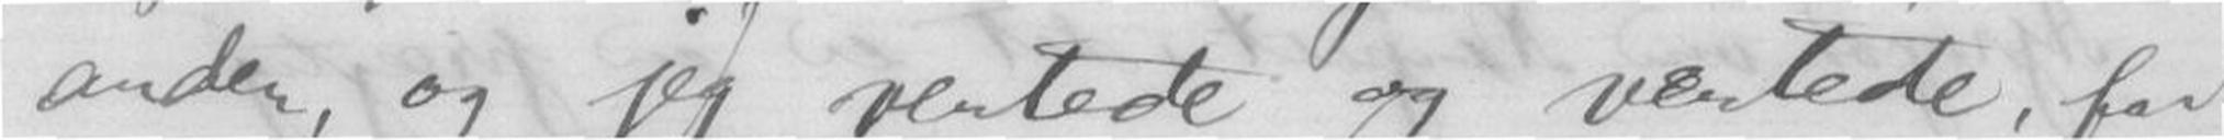anden, og jeg ventede og ventede, for
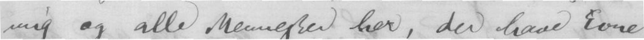mig og alle Mennesker her, der have Evne
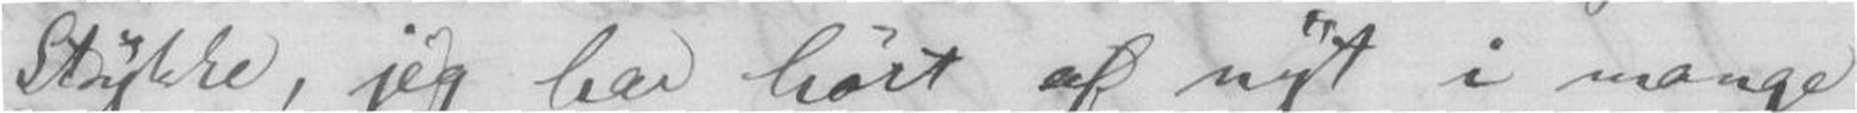Stykke, jeg har hørt af nyt i mange
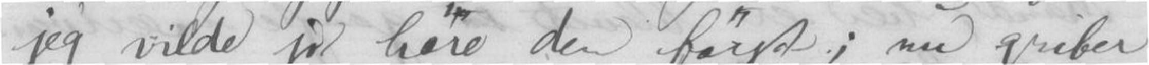jeg vilde jo høre den først; nu griber
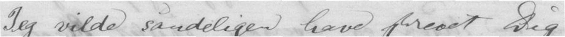Jeg vilde sandeligen have skrevet Dig
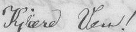Kjære Ven!
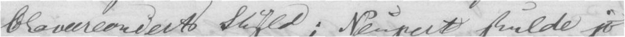Claverconserts Skyld; Neupert skulde jo
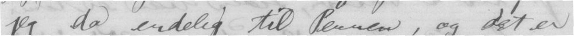jeg da endelig til Pennen, og det er
